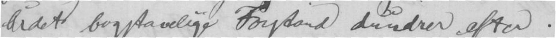Ordets bogstavelige Forstand dundrer efter.
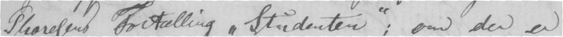Thoresens Fortælling Studenten; og der er
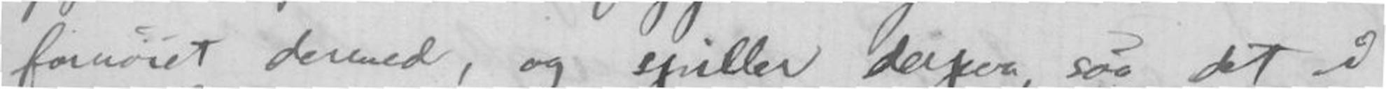fornøiet dermed, og spiller derpaa, saa det i
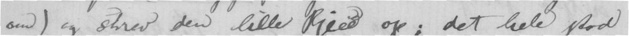om) og skrev den lille Pjece op; det hele stod
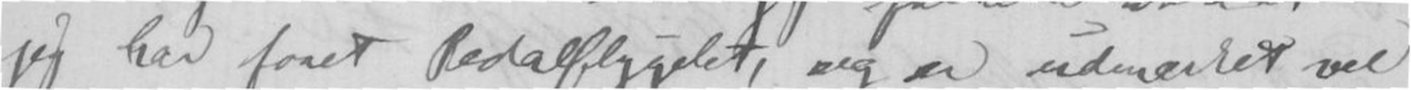jeg har tonet Pedalflygelet, og er udmærket vel
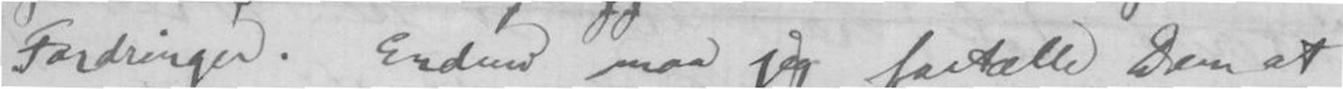Fordringer. Endnu maa jeg fortælle Dem at
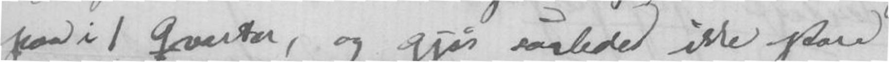paa i 1 quarter, og gjør saaledes ikke store
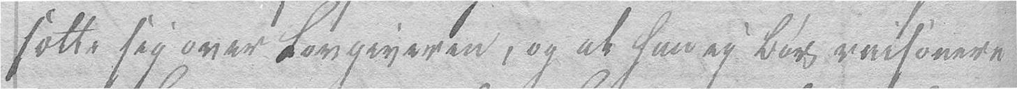sætte sig over Lovgiveren, og at han ey bør raisonnere
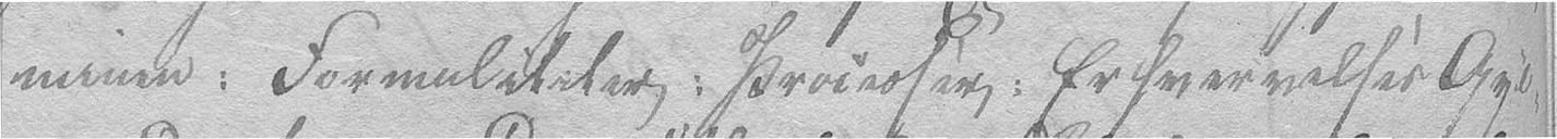mine: Formaliteter: Processer: Erhvervelses Gyl-
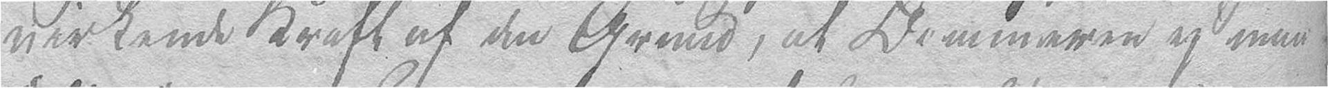virkende Kraft af den Grund, at Dommeren ey maa
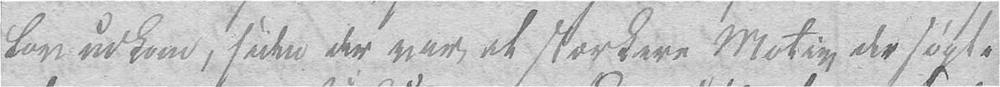Lov udkom, siden der var et stærkere Motiv der søgte
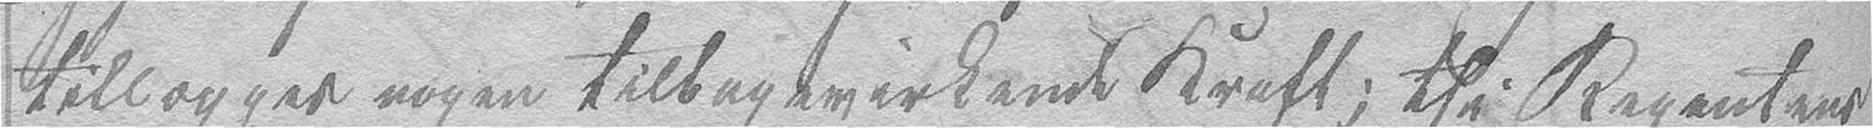tillægges nogen tilbagevirkende Kraft, thi Regentens
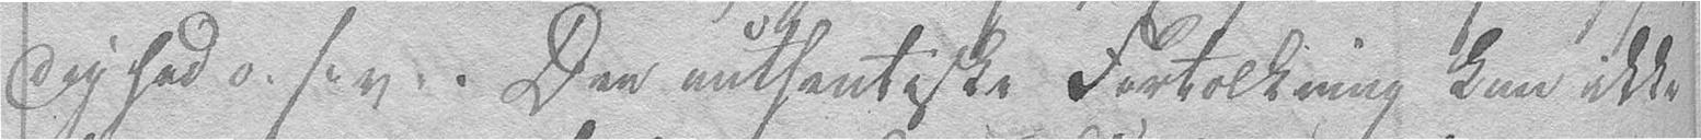dighed o.s.v. Den authentiske Fortolkning kan ikke
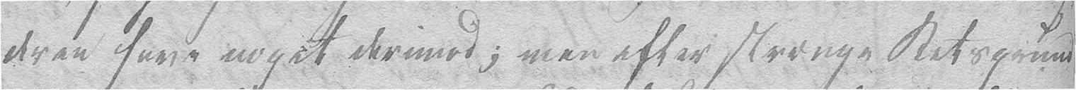deren have noget derimod, men efter strænge Retsgrund-
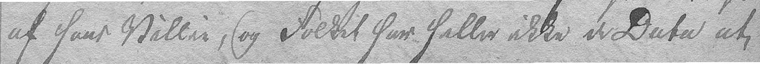af hans Villie, og Folket har heller ikke de Data at
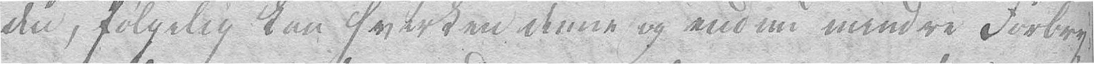den, følgelig kan hverken denne og endnu mindre Forbry-
